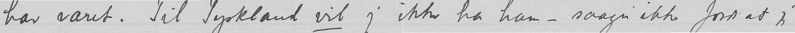har været.  Til Tyskland vil jeg ikke ha ham - saagar ikke fordi at jeg
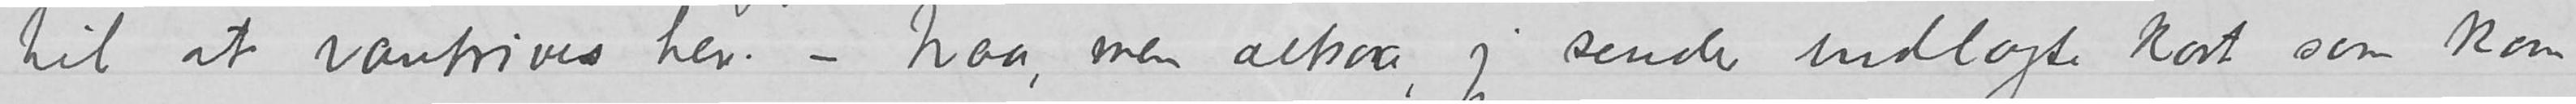til at vantrives her. - Naa, men altsaa, jeg sender indlagte kort som kom
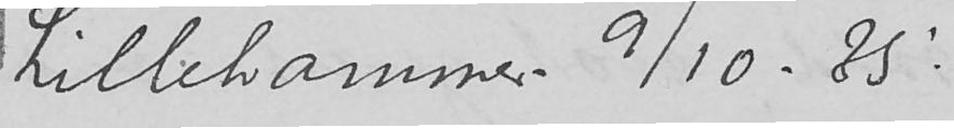Lillehammer 9/10-35.
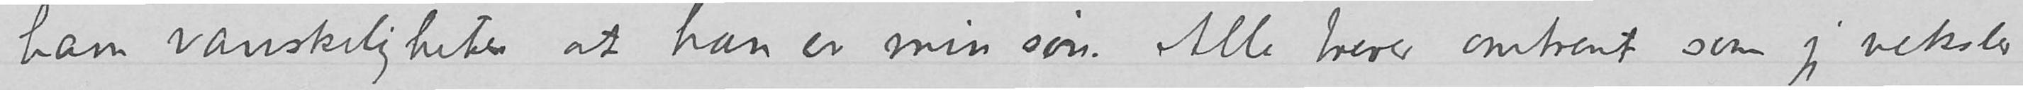ham vanskeligheter at han er min søn. Alle brever omtrent som jeg veksler
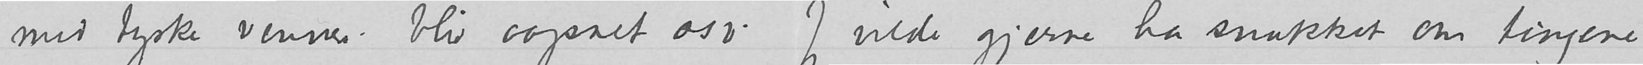med tyske venner blir aapnet osv. Jeg vilde gjerne ha snakket om tingene
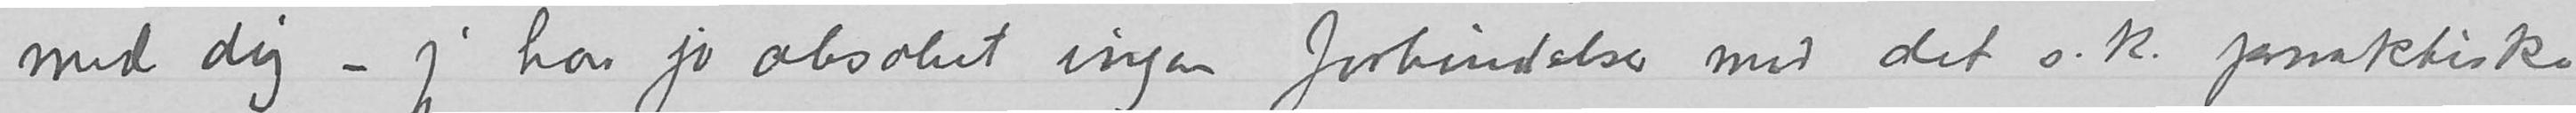med dig – jeg har jo absolut ingen forbindelse med det s.k. praktiske
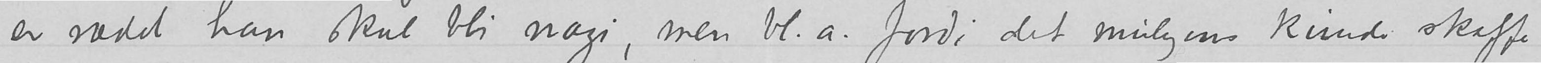er rædd han skal bli nazi, men bl.a. fordi det muligens kunde skaffe
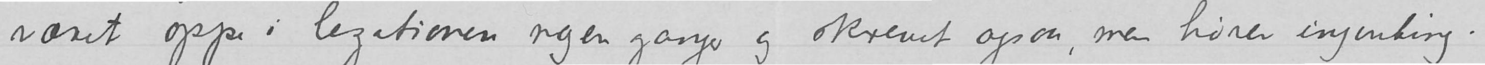været oppe i legationen nogen ganger og skrevet ogsaa, men hører ingenting.
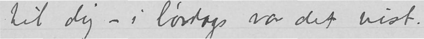til dig – i lørdags var det vist.
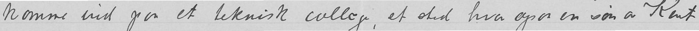komme ind paa et teknisk college, et sted hvor ogsaa en søn av Kent
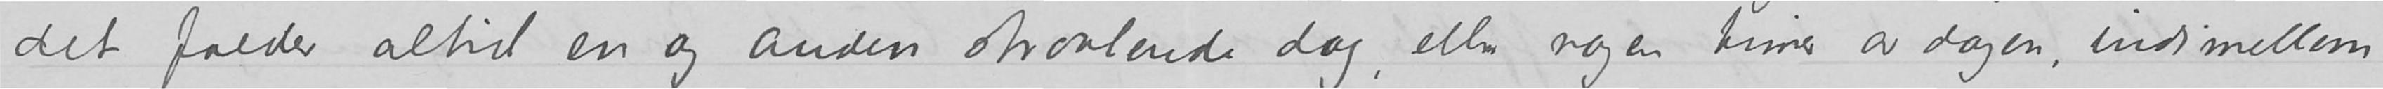det falder altid en og anden straalende dag, eller nogen timer av dagen, indimellem.
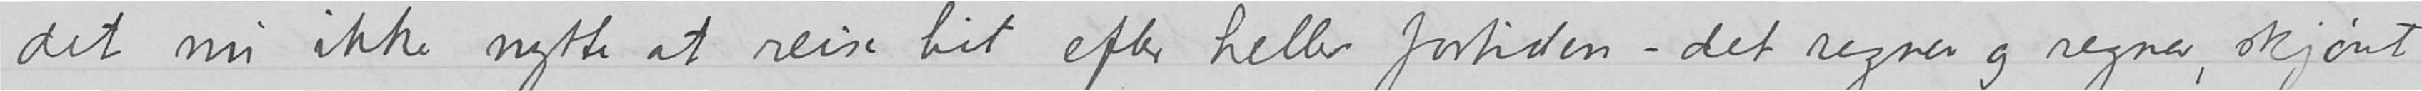det nu ikke nytte at reise hit efter heller fortiden – det regner og regner, skjønt
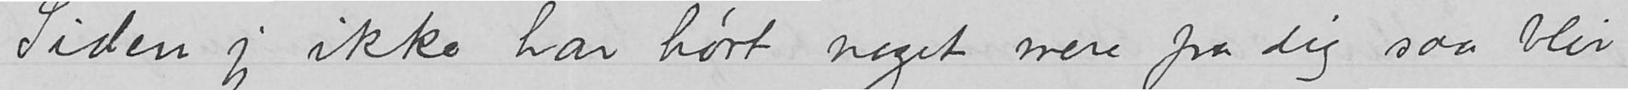Siden jeg ikke har hørt noget mere fra dig saa blir
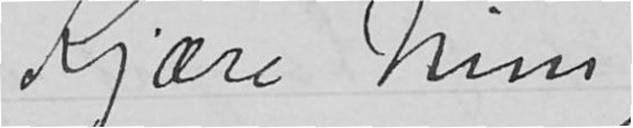Kjære Nini,
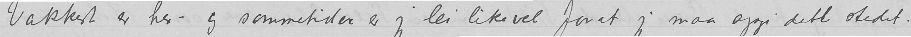Vakkert er her – og sommetider er jeg lei likevel for at jeg maa oppi dette stedet. til at vantrives her.  - Naa, men altsaa, jeg sender indlagte kort som kom til dig – i lørdags var det vist.
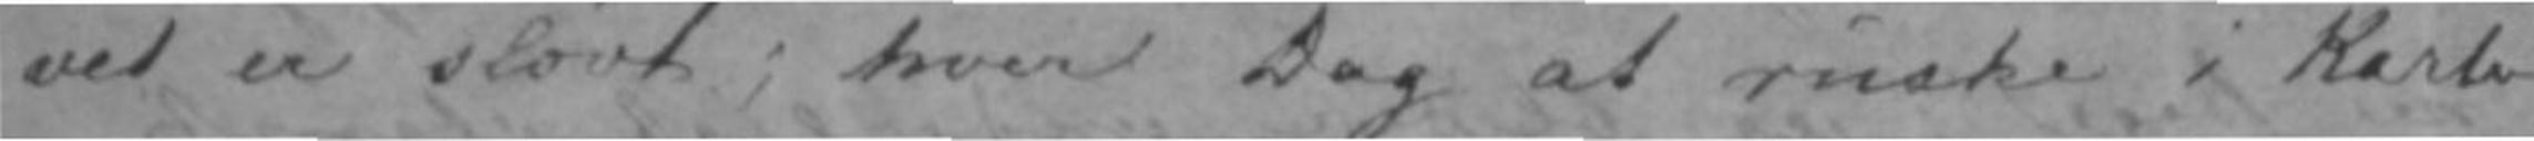vet er sløvt; hver Dag at ruske i Karle-
